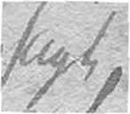Agt
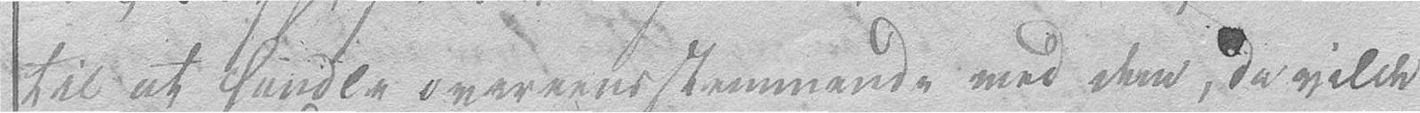til at handle overeensstemmende med dem, da vilde
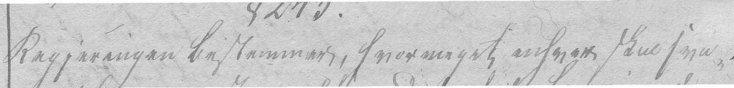Regjeringen bestemmer, hvormeget enhver skal sva-
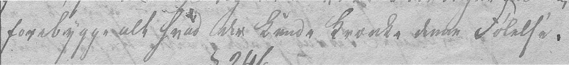forebygge alt hvad der kunde krænke denne Følelse.
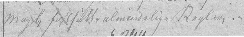Magt fastsatte almindelige Regler. –
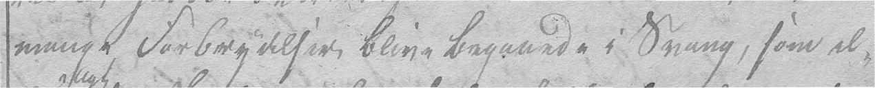mange Forbrydelser blive begaaede i Svang, som el-
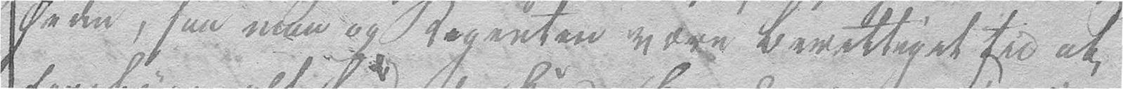heden, saa maa og Regenten være berettiget til at
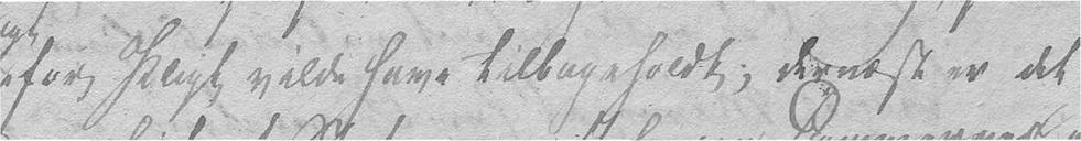for Pligt vilde have tilbageholdt; dernæst er det
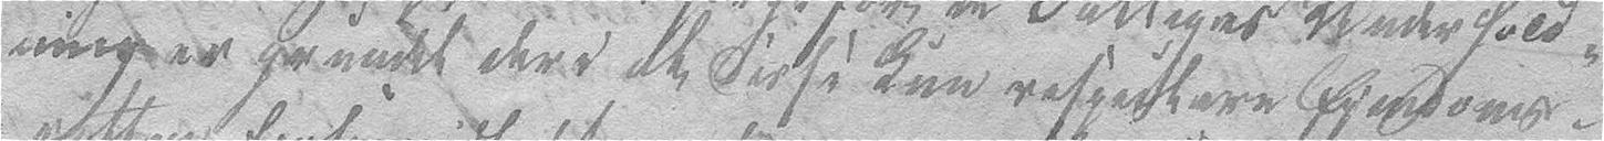ning er grundet deri at disse kun respectere Eiendoms-
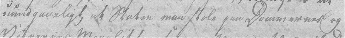uundgaaeligt at Staten maa stole paa Dommernes og
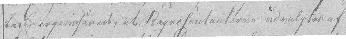ledes organiserede, at Repræsentanterne udvalgtes af
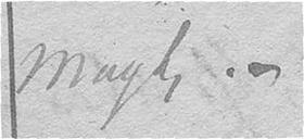Magt. –
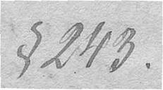§ 243.
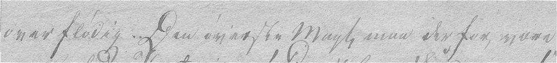overflødig. Den øverste Magt maa derfor være
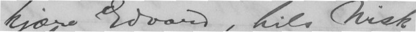kjære Edvard, hils Nisk
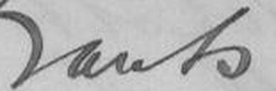Frants.
